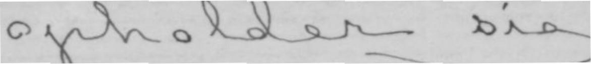opholder sig.
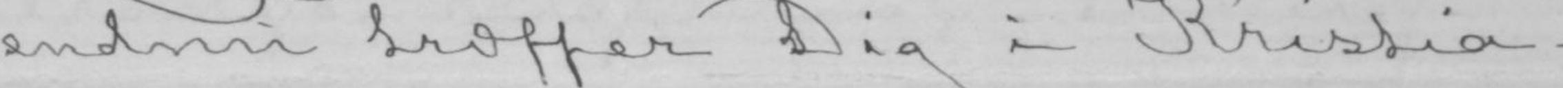endnu træffer Dig i Kristia-
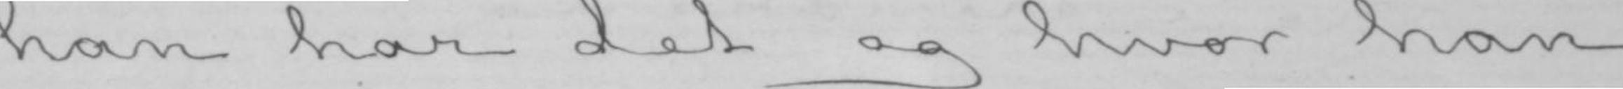han har det og hvor han
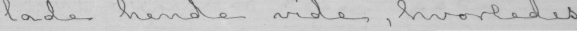lade hende vide, hvorledes
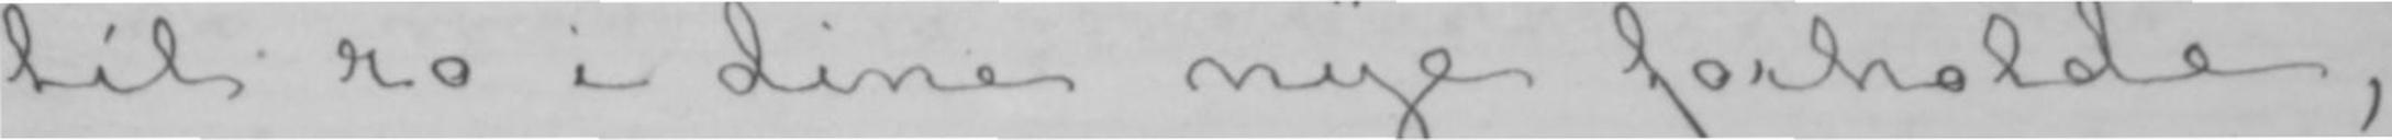til ro i dine nye forholde,
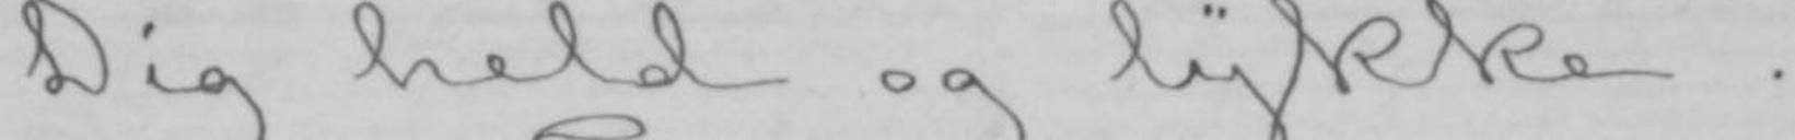Dig held og lykke.
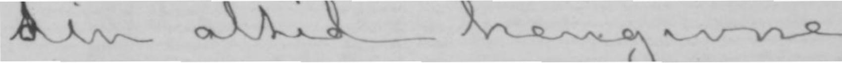din altid hengivne
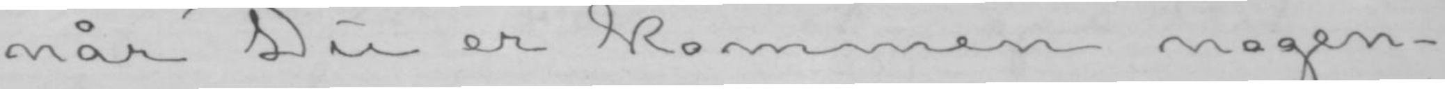når Du er kommen nogen-
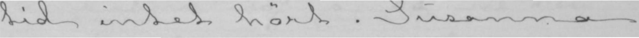tid intet hørt. Susanna
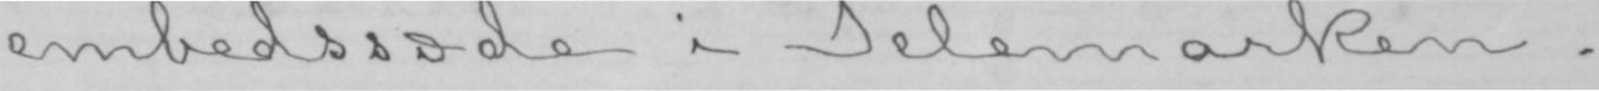embedssæde i Telemarken.
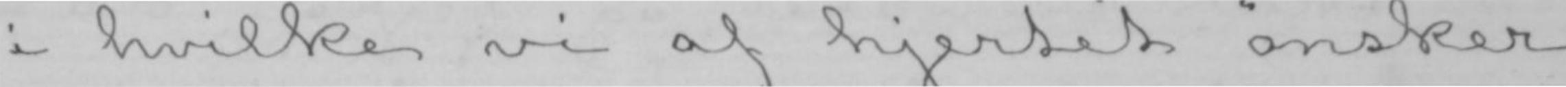i hvilke vi af hjertet ønsker
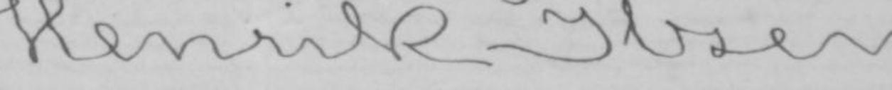Henrik Ibsen.
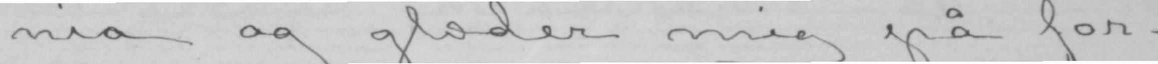nia og glæder mig på for-
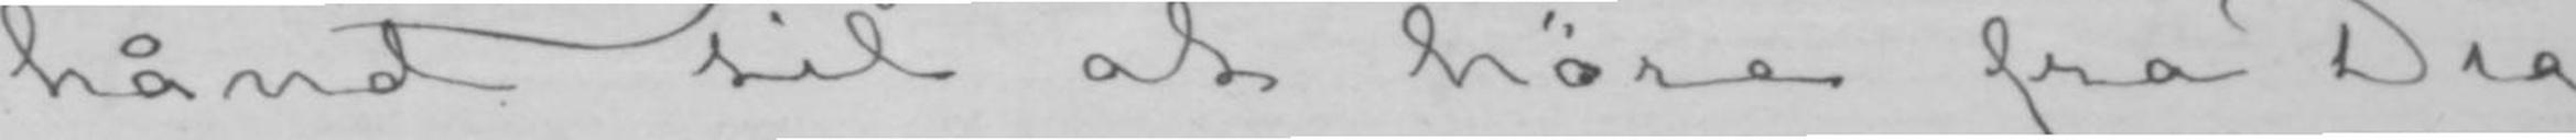hånd til at høre fra Dig
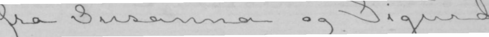fra Susanna og Sigurd
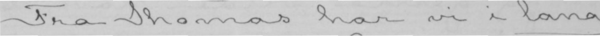Fra Thomas har vi i lang
